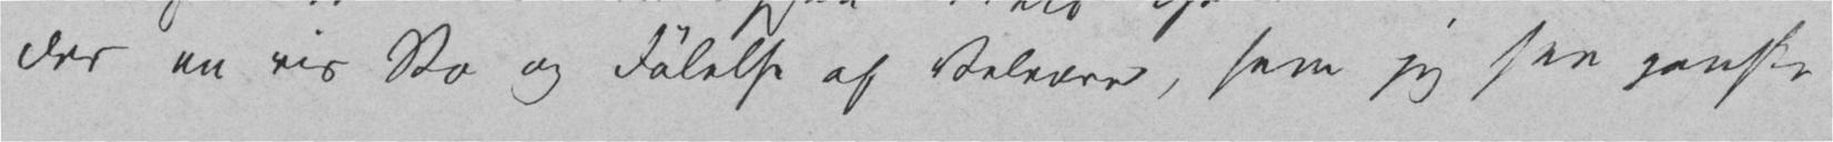der en vis Ro og Følelse af Velvære, som jeg saa ganske
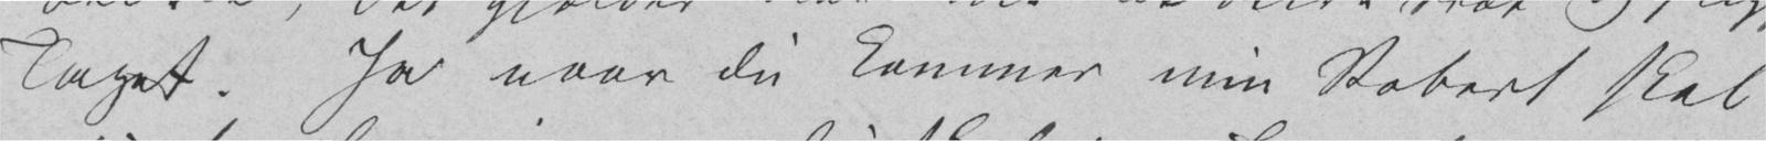Taget. Ja naar Du kommer min Robert skal
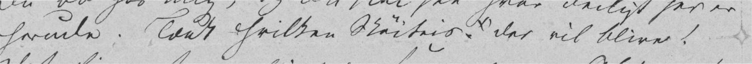herude. Tænk hvilken Skøiteis! der vil blive!
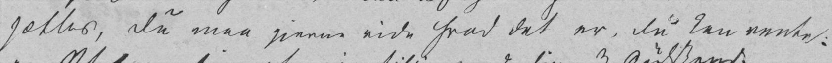sættes, Du maa gjerne vide hvad det er, Du kan vente:
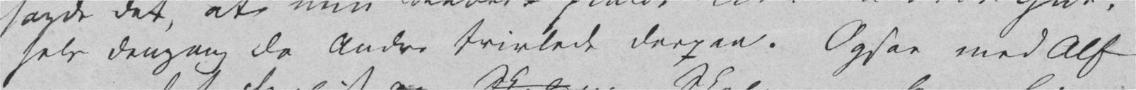selv dengang da Andre tvivlede derpaa. Ogsaa med Alf
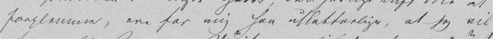forglemme, ere for mig saa uskatterlige, at jeg vil
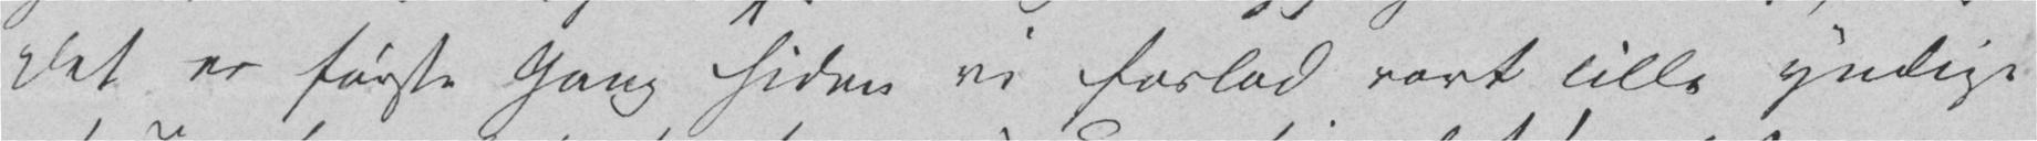det er første Gang siden vi forlod vort lille yndige
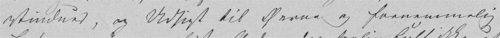Vinduer, og Udsigt til Øerne og fornemmelig
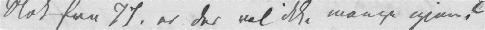Stok fra 77. er der vel ikke mange igjen?
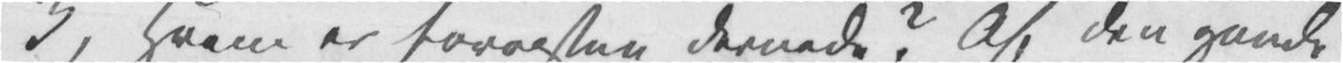3) Hvem er forresten dernede? Af den gamle
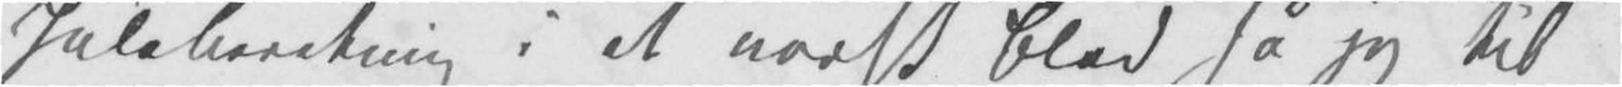Juleberetning i et norsk Blad så jeg til
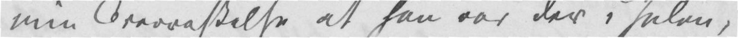min Overraskelse at han var der i Julen,
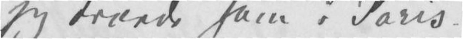jeg troede ham i Paris.
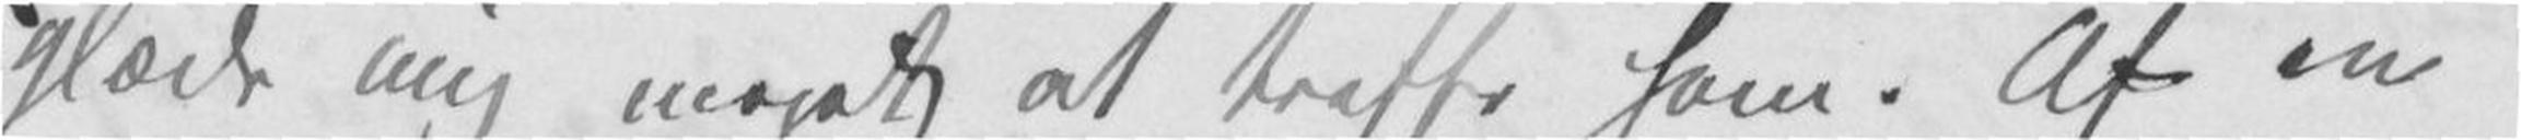glæde mig meget at treffe ham. Af en
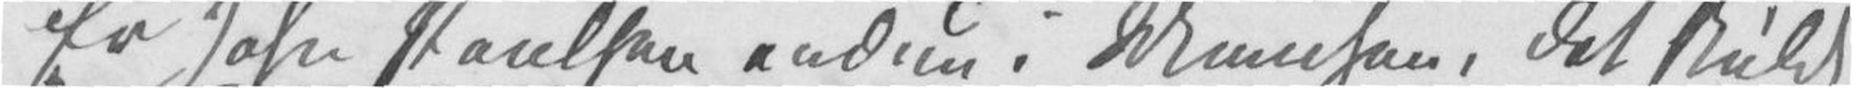Er John Paulsen endnu i München, det skulde
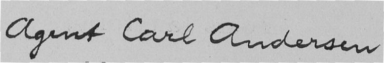Agent Carl Andersen
